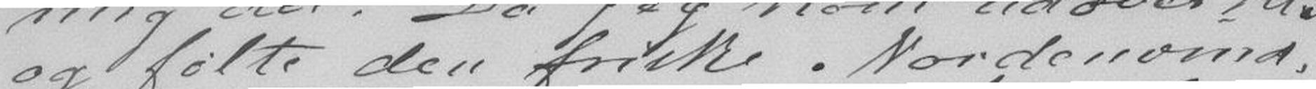og følte den friske Nordenvind,
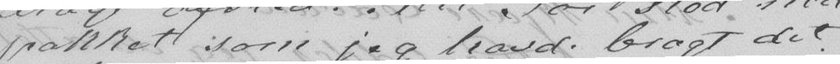pakket som jeg havde bragt det
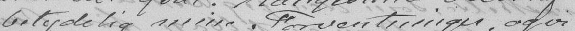betydelige mine Forventninger, og vi
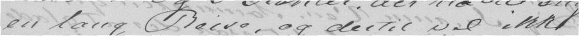en lang Reise, og dertil vel ikke
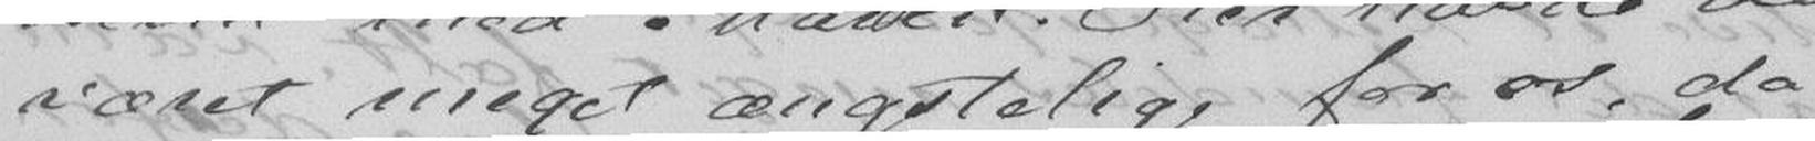været meget ængstelige, for os, da
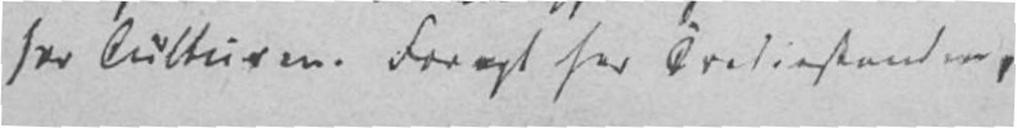for Culturen. Foragt for Traditionen,
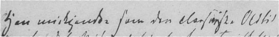Han miskjende, som den Classiske Oldtid
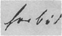forbød
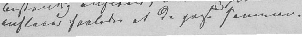anslaaer saaledes at de passe sammen.
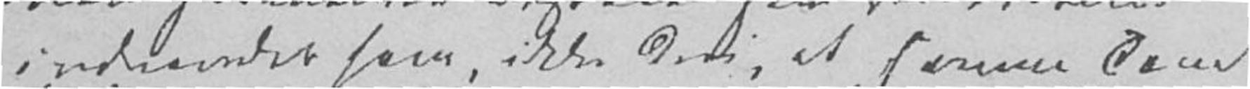indvender ham, ikke deri, at samme Tone
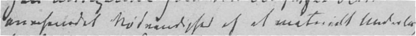overhovedet Nødvendighed af et materielt Underlag
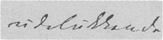udelukkende
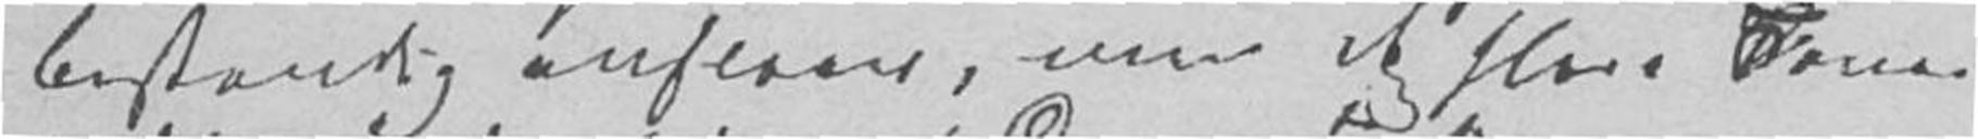bestandig anslaaer, men at flere Toner
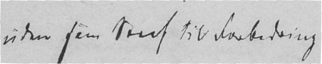uden som Straf til Forbedring
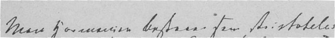Men Harmonien bestaaer som Aristoteles
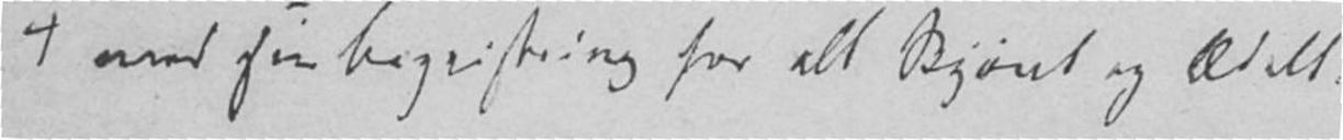med sin Begeistring for alt Skjønt og Ædelt.
